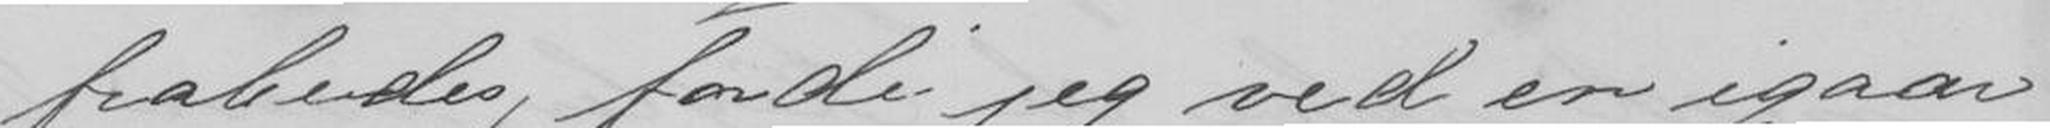frabedes, fordi jeg ved en igaar
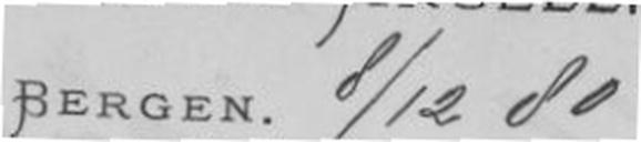BERGEN. 8/12 80
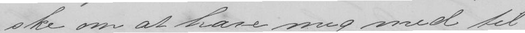ske om at have mig med til
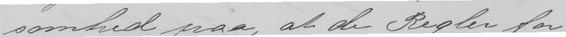somhed paa, at de Regler for
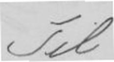Til
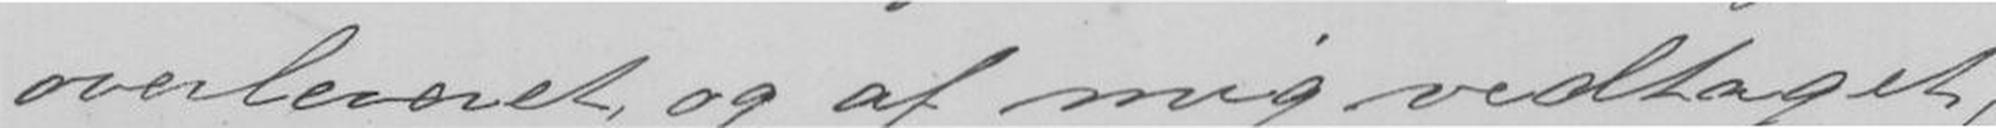overleveret og af mig vedtaget,
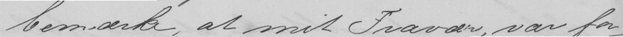bemærke, at mit Fravær, var for-
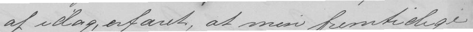i dag erfaret, at min fremtidige
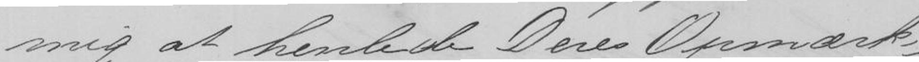mig at henlede Deres Opmærk-
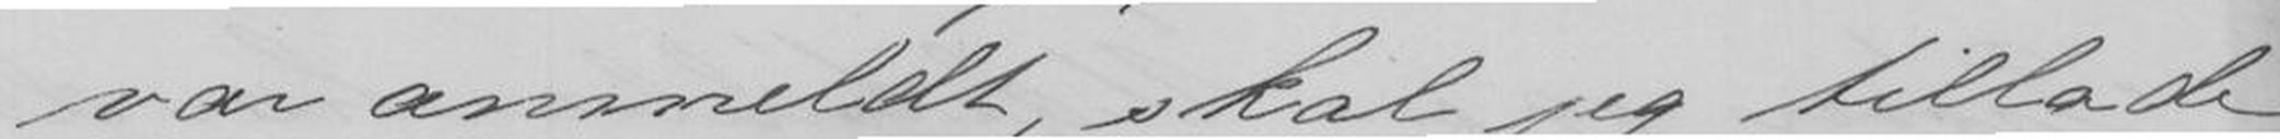var anmeldt, skal jeg tillade
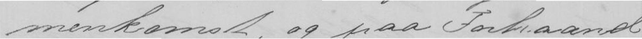menkomst, og paa Forhaand
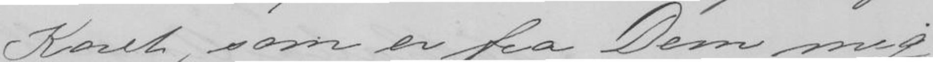Koret, som er fra Dem mig
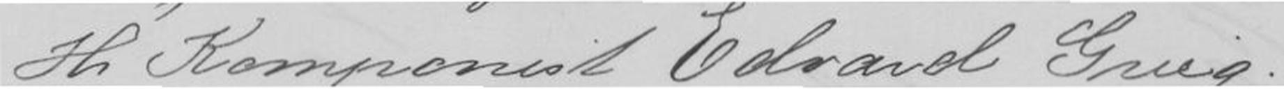Hr Komponist Edvard Grieg.
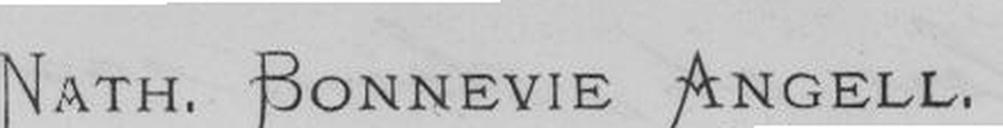NATH. BONNEVIE ANGELL
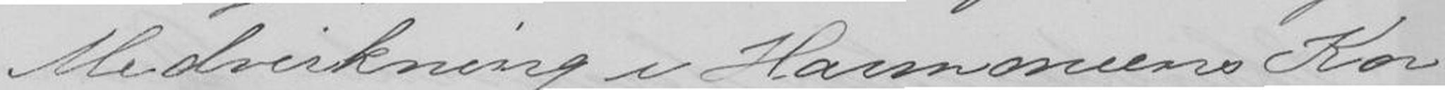Medvirkning i Harmoniens Kor
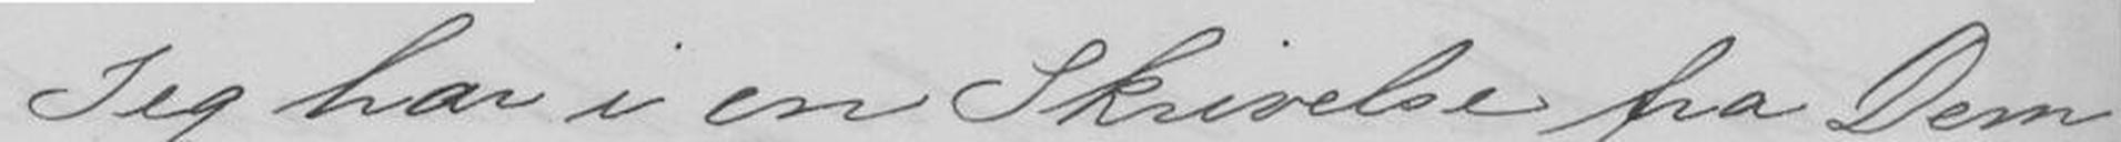Jeg har i en Skrivelse fra Dem
